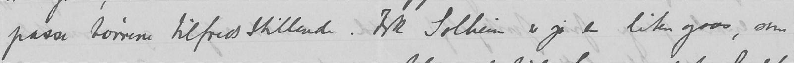passe børnene tilfredsstillende.  Frk Solheim er jo en liten gaas, som
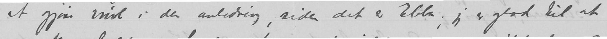at gjøre virvl i den anledning, siden det er Ebba; jeg er glad til at
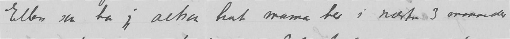Ellers saa har jeg altsaa hat mama her i næsten 3 maaneder
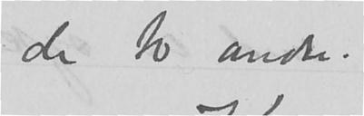de to andre.
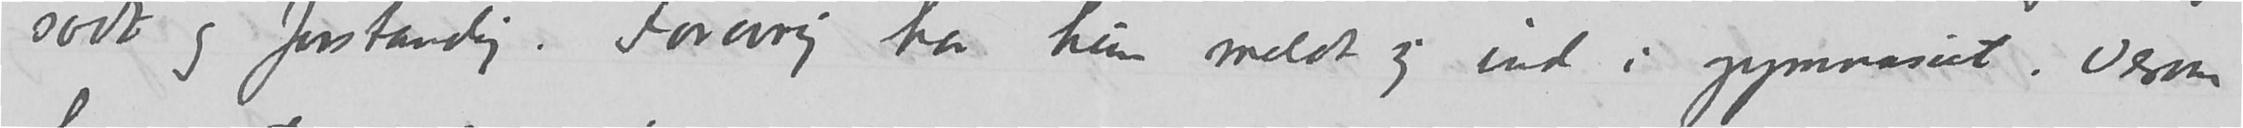sødt og forstandig. Forøvrig har hun meldt sig ind i gymnasiet.  Derom
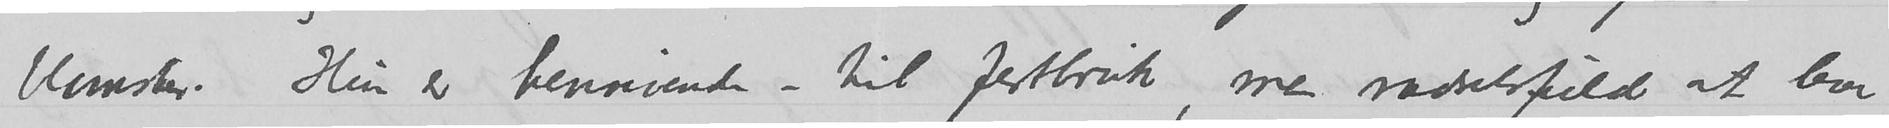blomster.  Hun er henrivende – til festbruk, men rædselsfuld at leve
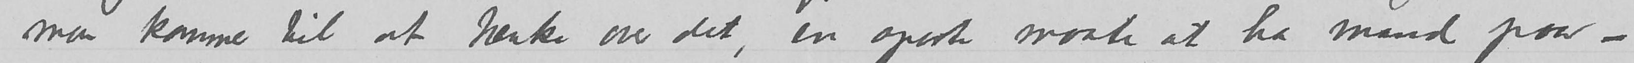man kommer til at tænke over det, en aparte maate at ha mand paa –
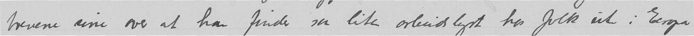brevene sine over at han finder saa lite arbeidslyst hos folk ute i Europa
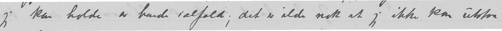jeg kan holde av hende ialfald; det er ilde nok at jeg ikke kan utstaa
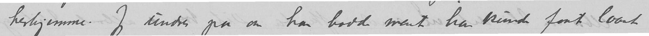herhjemme. Jeg undres paa om han <hadde> ment han kunde faat <laant>
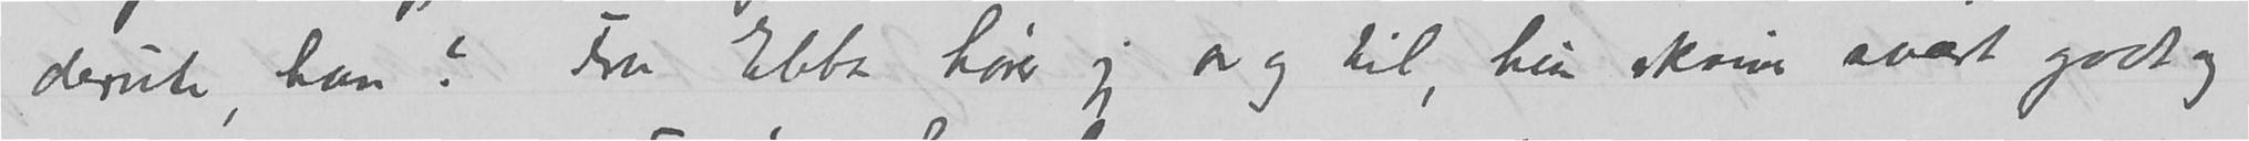derute, han?  Fra Ebba hører jeg av og til, hun skriver svært godt og
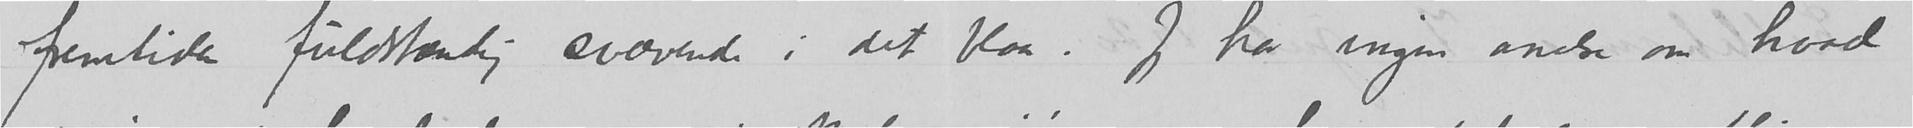fremtiden fuldstændig svævende i det blaa.  Jeg har ingen anelse om hvad
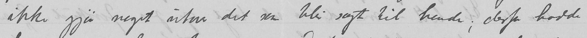ikke gjør meget utover det som blit sagt til hende; derfor hadde
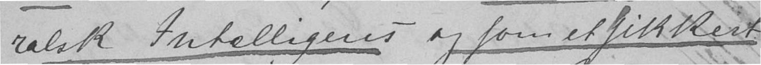ralsk Intelligens og som et sikkert
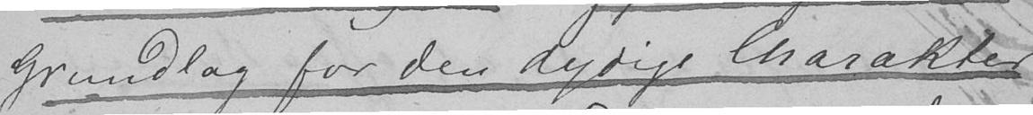Grundlag for den dydige Charakter.
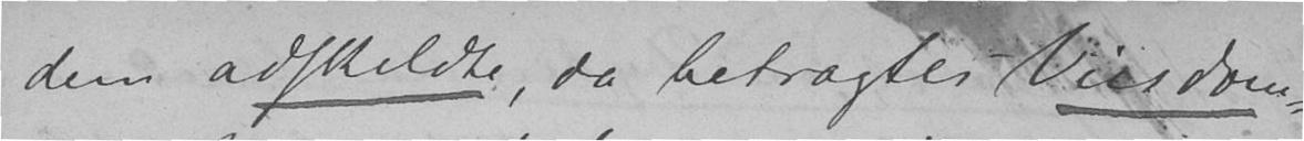dem adskildte, da betragtes Visdom-
